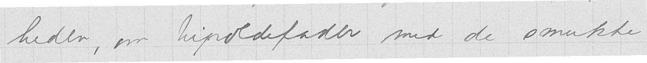heden, om tipoldefader med de smukke
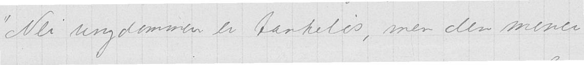"Nei ungdommen er tankeløs, men den mener
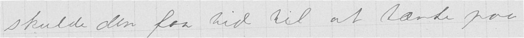skulde den faa tid til at tænke paa
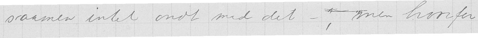saamen intet ondt med det -, men hvorfor
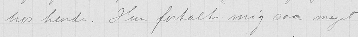hos hende. Hun fortalte mig saa meget
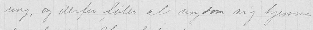ung, og derfor føler al ungdom sig hjemme
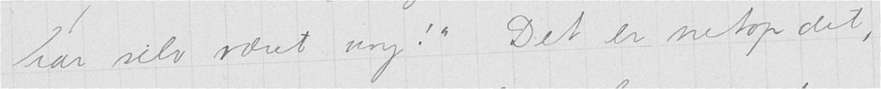har selv været ung!" Det er netop det,
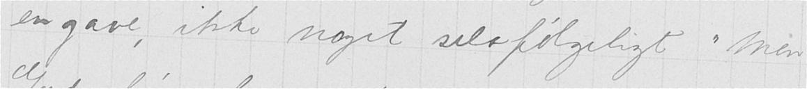en gave, ikke noget selvfølgeligt. "Men
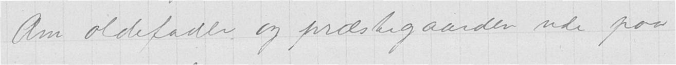Om oldefader og præstegaarden ude paa
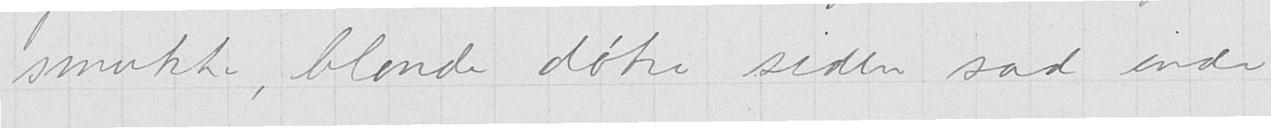smukke, blonde døtre siden sad inde
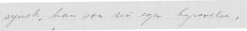synsk, han saa sin egen begravelse,
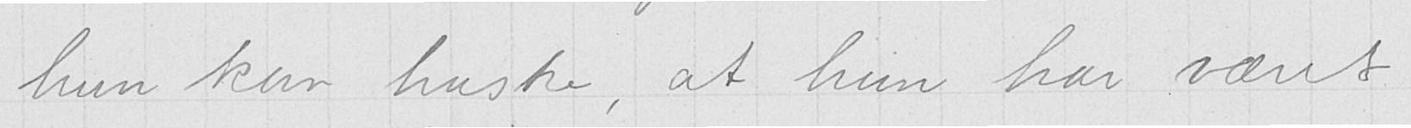hun kan huske, at hun har været
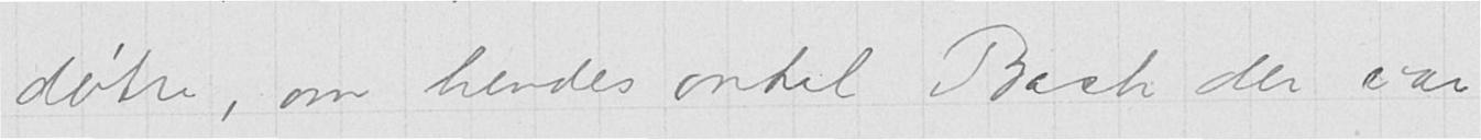døtre, om hendes onkel Bech der var
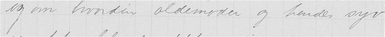og om hvordan oldemoder og hendes syv
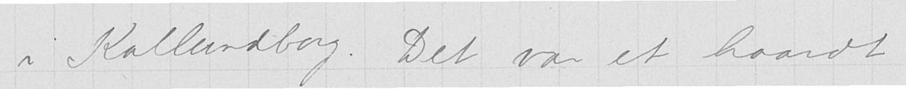i Kallundborg. Det var et haardt
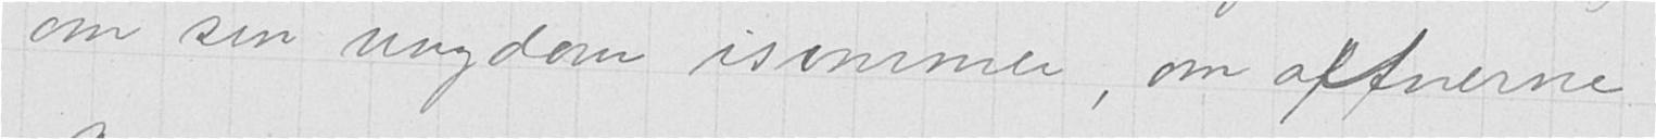om sin ungdom i sommer, om aftnerne.
